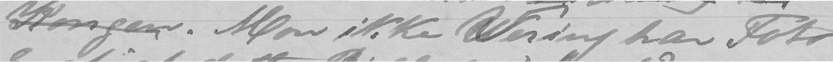Kongen. Mon ikke Wering har Foto-
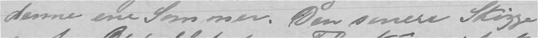denne ene Sommer. Den senere Skizze
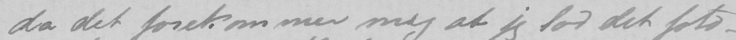da det forekommer mig at jeg lod det foto-
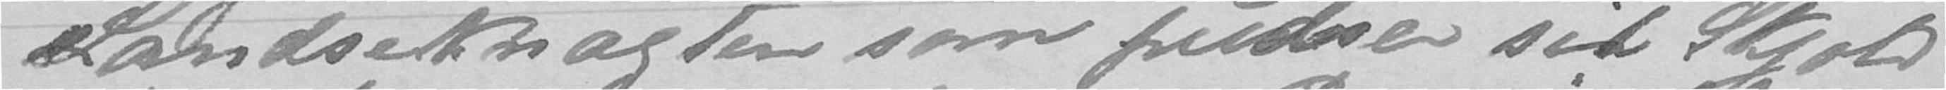Landseknægten som pudser sid Skjold
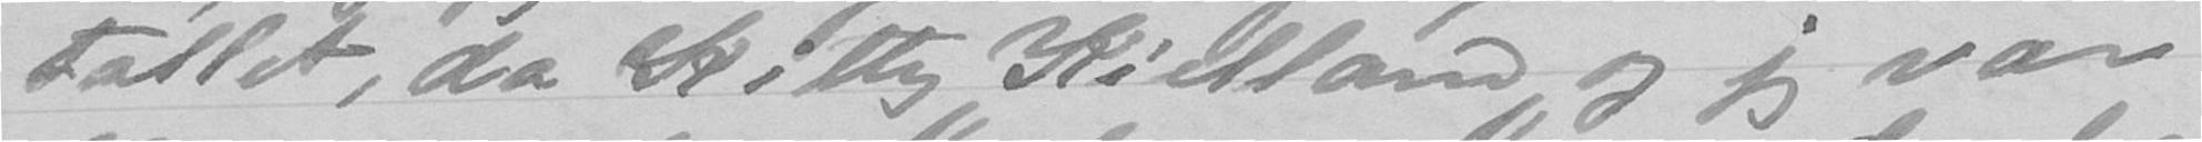tallet, da Kitty Kielland og jeg var
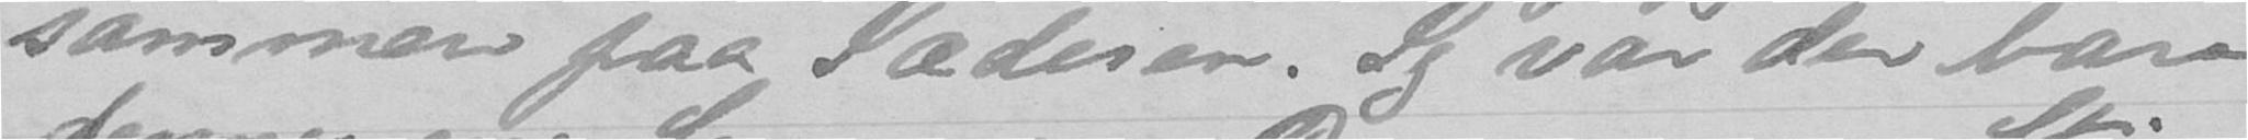sammen paa Sæderen. Jeg var der bare
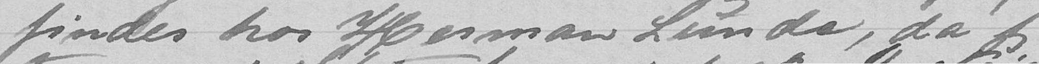findes hos Herman Lunde, da jeg
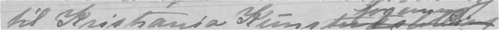til Kristiania Kunstudstilling
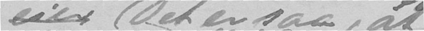eier Det er saa, at
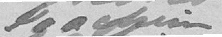Joachim
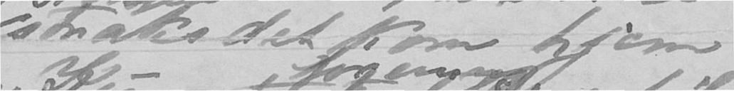straks det kom hjem
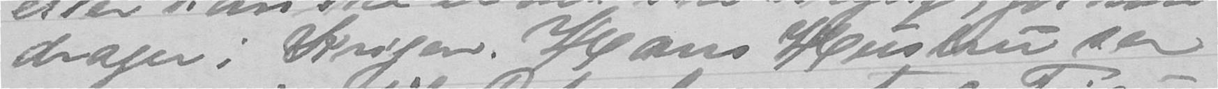drager i Krigen. Hans Hustru ser
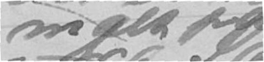malt fra
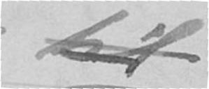til
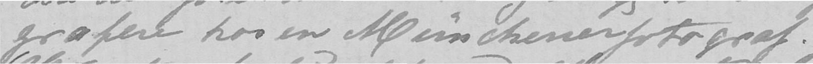grafere hos en Münchenerfotograf.
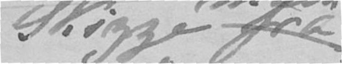Skizze fra
